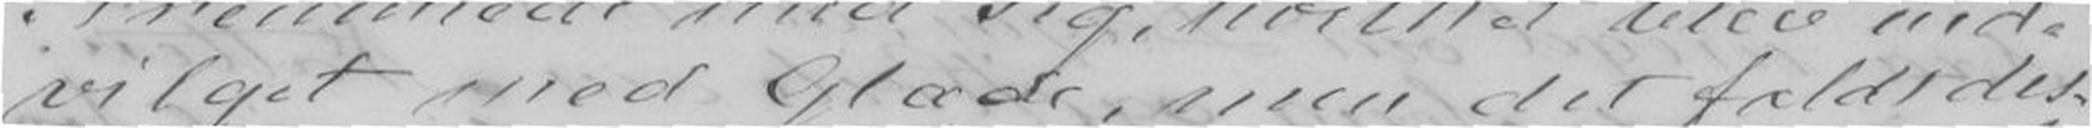vilgat med Glæde, men det faldt des-
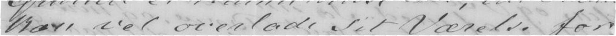kan vel overlade sit Værelse for
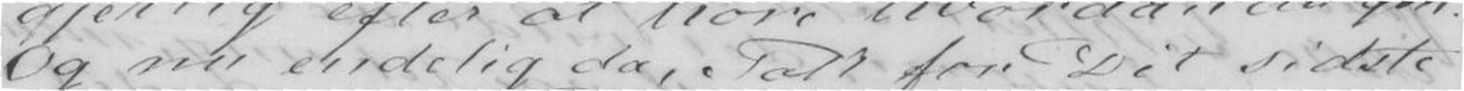Og nu endelig da, Tak for Dit sidste
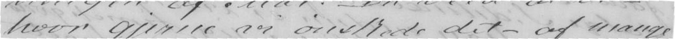hvor gjerne vi ønskede det - af mange
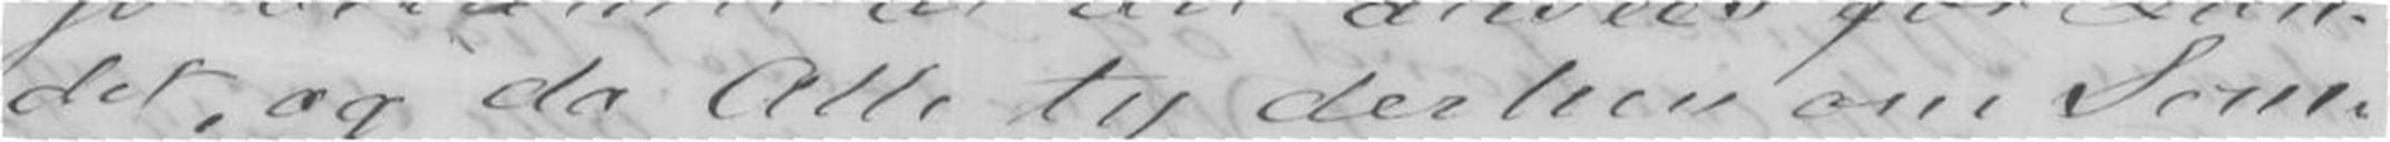det, og da Alle ty der hen om Som-
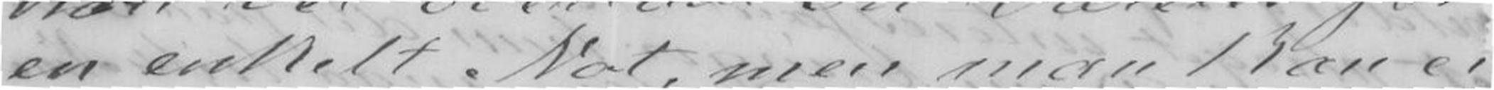en enkelt Nat, men man kan ei
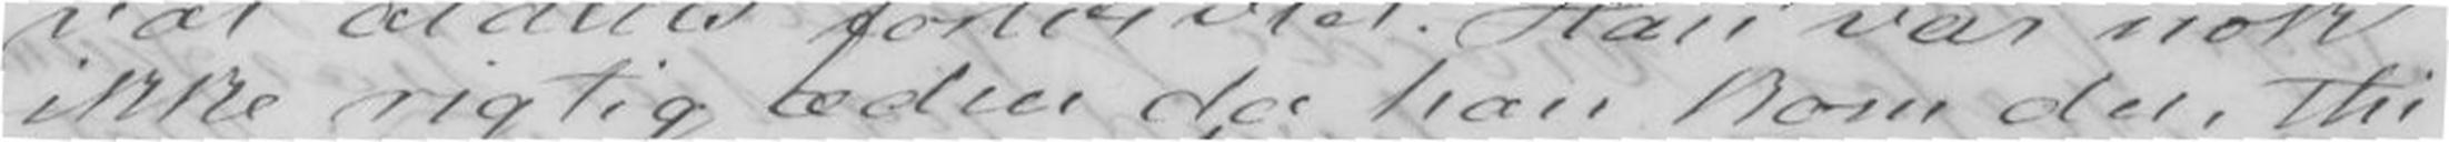ikke rigtig ædru da han kom der, thi
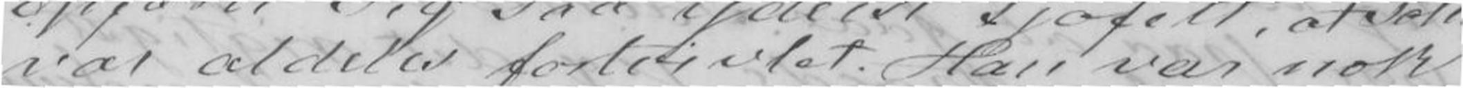var aldeles fortvivlet. Han var nok
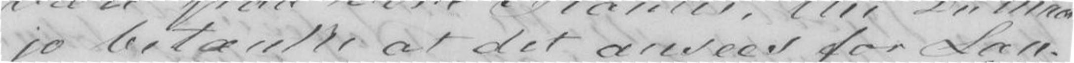jo betænke at det ansees for Lan-
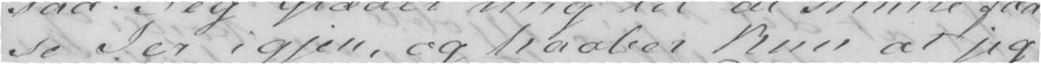se Jer igjen, og haaber kun at jeg
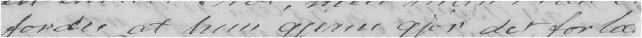fordre at hun gjerne gjør det for læ
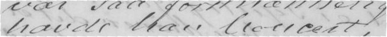havde han Concert,
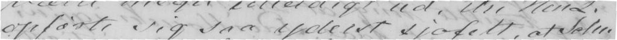opførte sig saa yderst sjofelt, at John
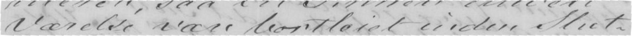Værelse være bortleiet inden Slut-
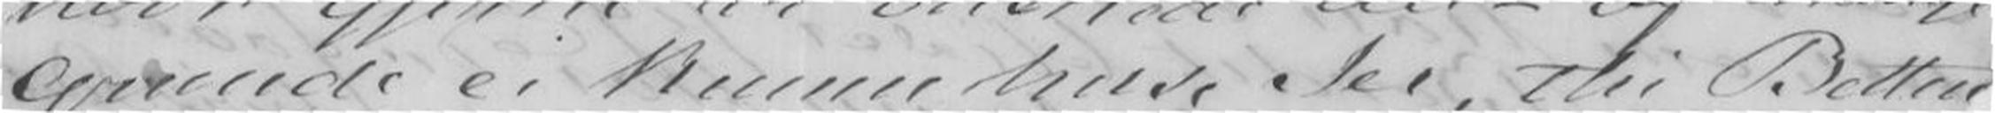Grunde ei kunne huse Jer, thi Betten
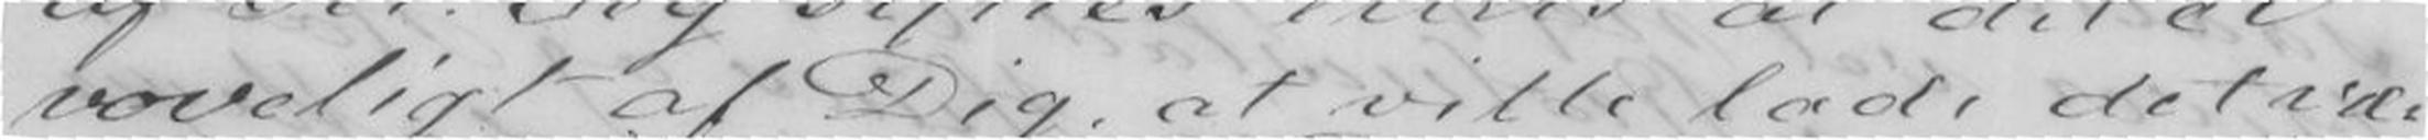voveligt af Dig at ville lade det væ-
